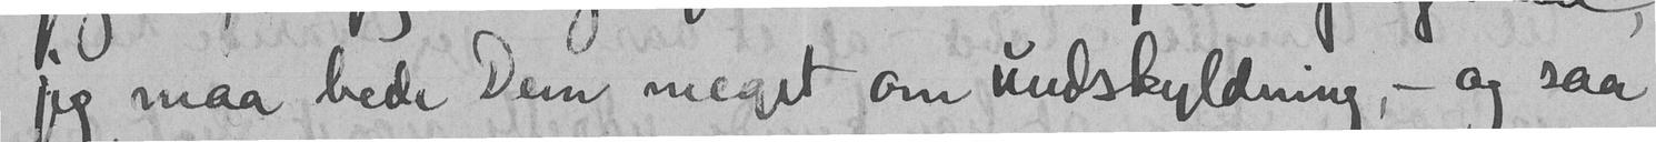jeg maa bede Dem meget om undskyldning, - og saa
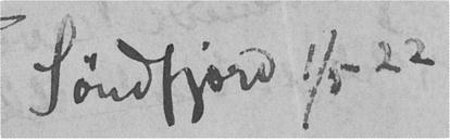Søndfjord 1/5 22
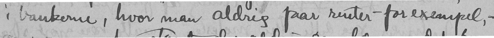i bankerne, hvor man aldrig faar renter - for exempel,-
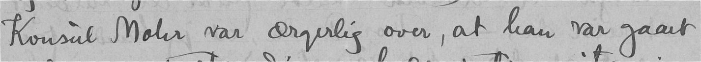Konsul Mohr var ærgerlig over, at han var gaaet
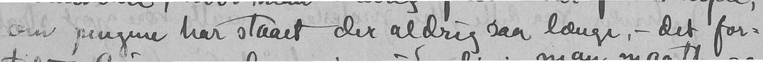om pengene har staaet der aldrig saa længe, - det for-
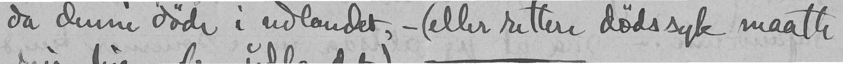da denne døde i udlandet, - (eller rettere dødssyk maatte
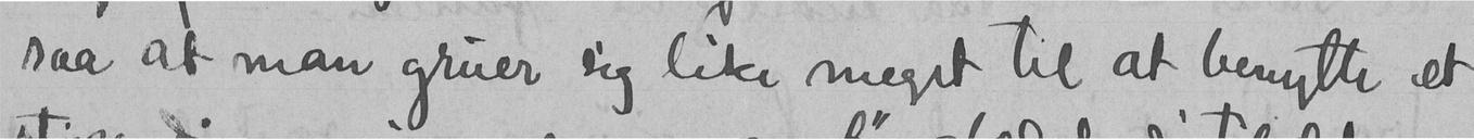saa at man gruer sig like meget til at benytte et
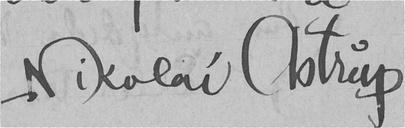Nikolai Astrup
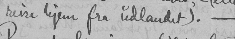reise hjem fra udlandet). -
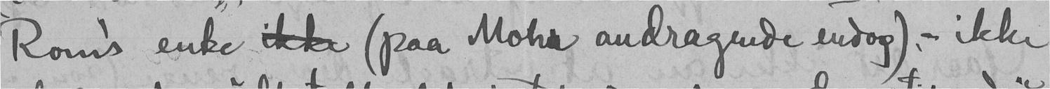Rom's enke  (paa Mohr andragende endog), - ikke
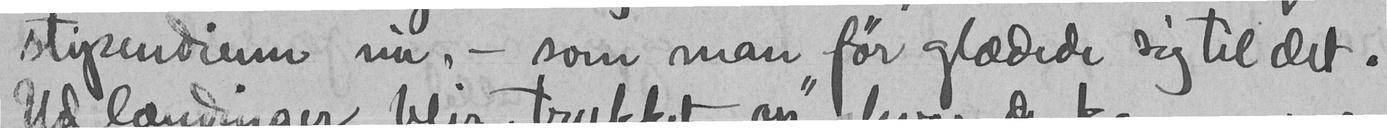stipendium nu, - som man før glædede sig til det.
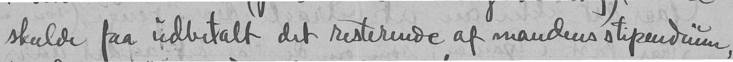skulde faa udbetalt det resterende af mandens stipendium,
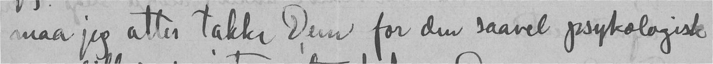maa jeg atter takke Dem for den saavel psykelogisk
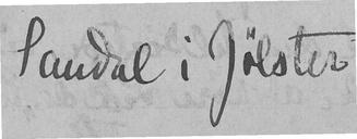Sandal i Jølster
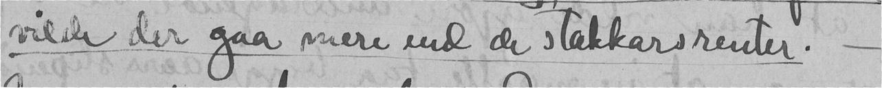vilde der gaa mere end de stakkars renter. -
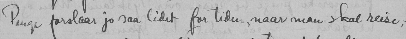Penge forslaar jo saa lidet for tiden, naar man skal reise, -
